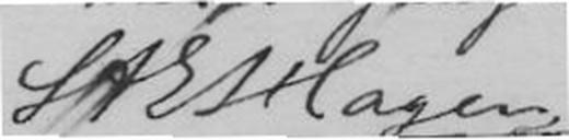S A E Hagen
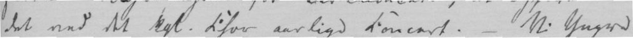det ved det kgl Chor aarlige Concert. - Vi Ungre
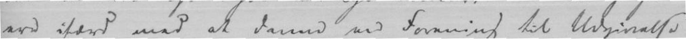ere ifærd med at danne en Forening til Idgivelse
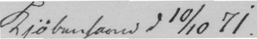Kjøbenhavn d 10/10 71.
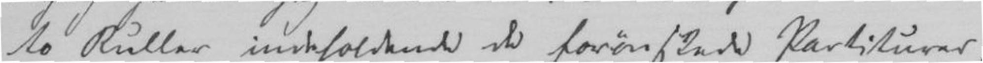to Ruller indeholdende de forønskede Partiturer,
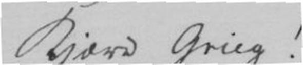Kjære Grieg!
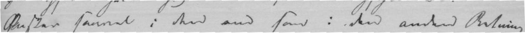Ønsker saavel i den ene som i den anden Retning-
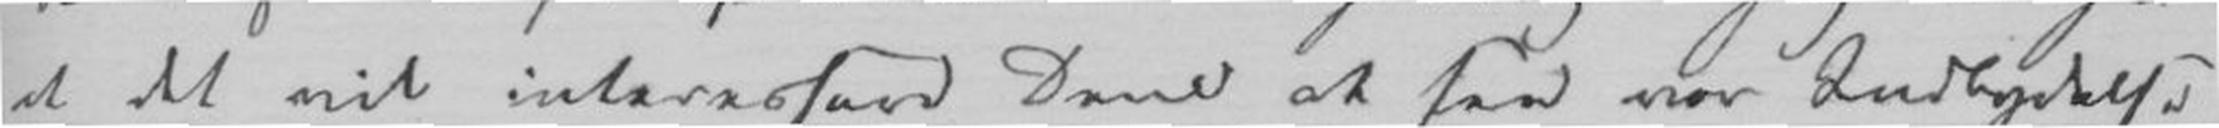at det vil interessere Dem at see vor Indbydelse
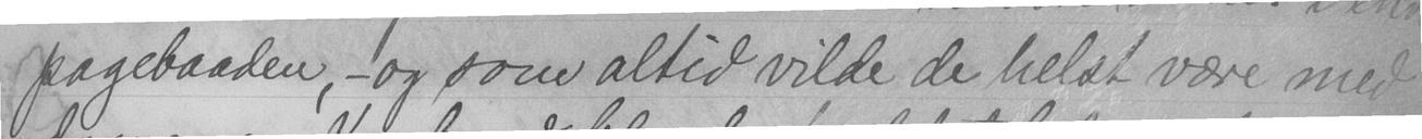pagebaaden, - og som altid vilde de helst være med
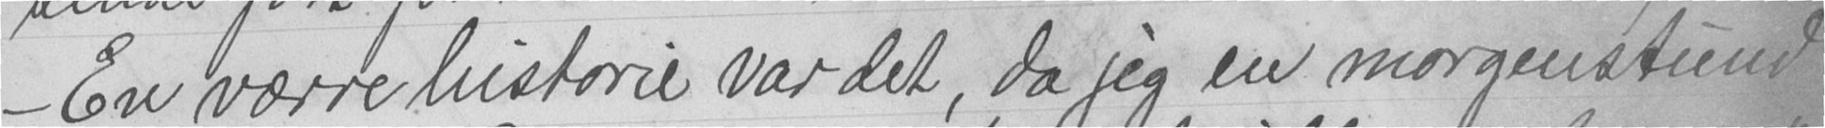- En værre historie var det, da jeg en morgenstund
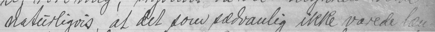naturligvis, at det som sædvanlig ikke varede læn-
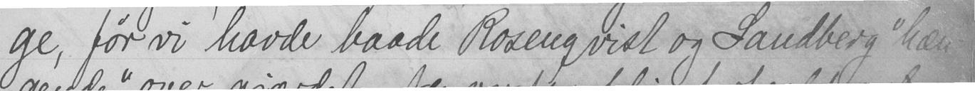ge, før vi havde baade Rosenqvist og Sandberg "hæn
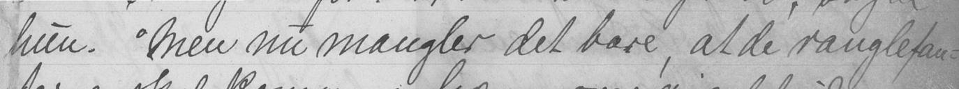hun. "Men nu mangler det bare, at de ranglefan-
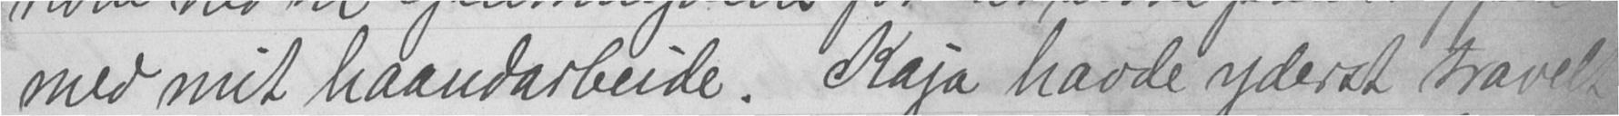med mit haandarbeide. Kaja havde yderst travelt-
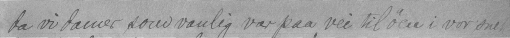da vi damer som vanlig var paa vei til øen i vor snek-
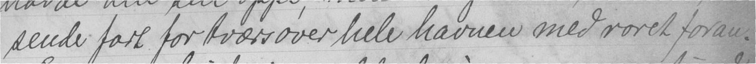sende fart for tværsover hele havnen med roret foran.
